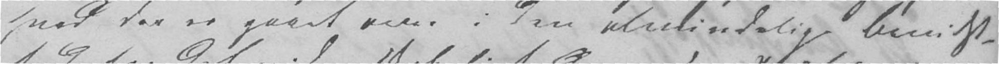hvad der er gaaet over i den almindelige Bevidst-
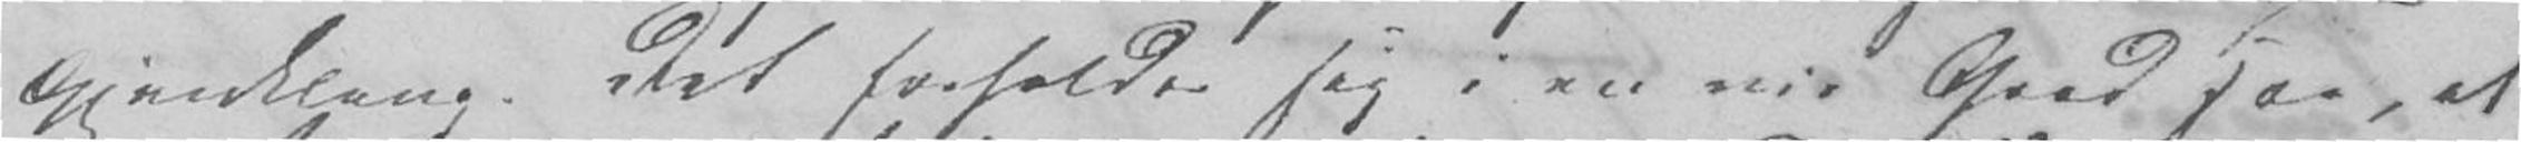Gjenklang. Det forholder sig i en vis Grad saa, at
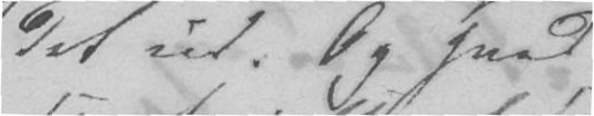det ud. Og hvad
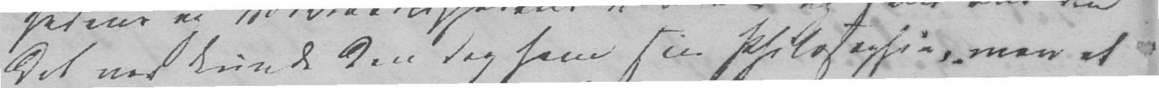det var kunde den dog have sin Philosophie, men at
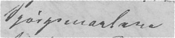Spørgsmaalene
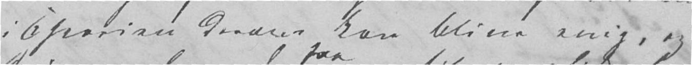i Theorien derom kan blive enig, og
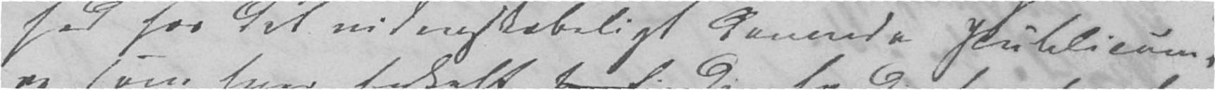hed hos det videnskabeligt dannede Publicum,
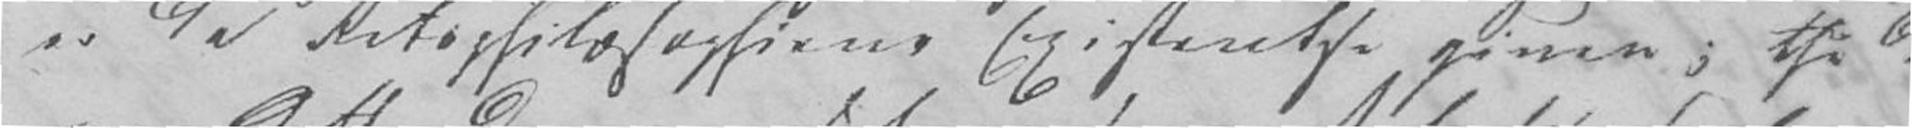er da Retsphilosophiens Existentse given; thi
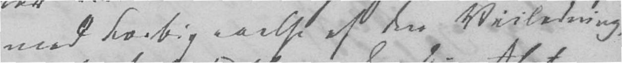med Forbigaaelse af den Veiledning,
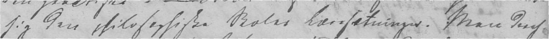sig den philosophiske Skoles Læresætninger. Men deraf
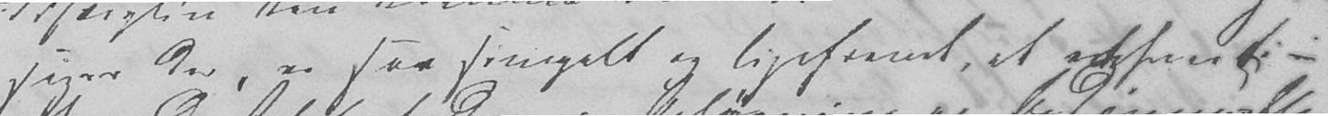siges der, er saa simpelt og ligefremt, at ethvert -
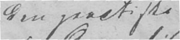den practiske
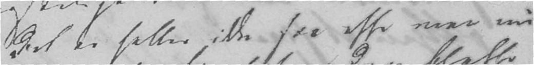Det er heller ikke saa ofte man nu
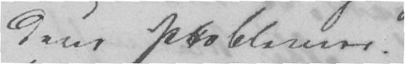dens Problemer.
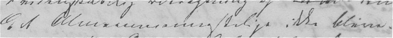det Almeenmenneskelige ikke blive.
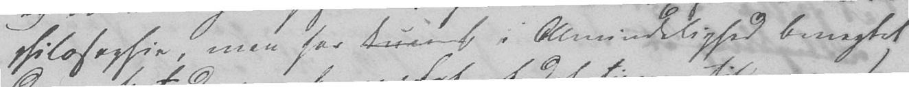philosophie, man har kun i Almindelighed benægtet
